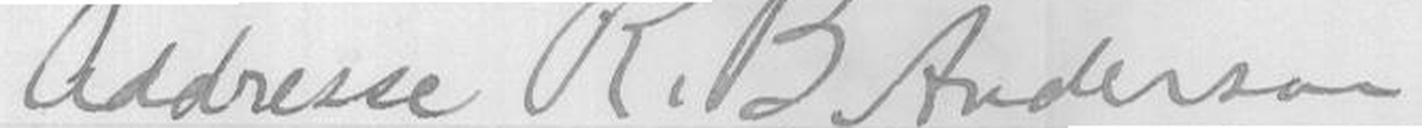Adresse R.B. Anderson
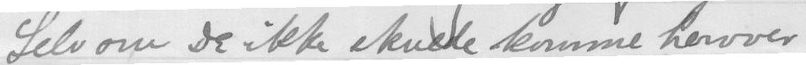Selv om De ikke skulde komme herover,
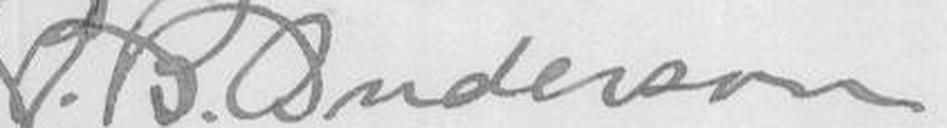R.B. Anderson
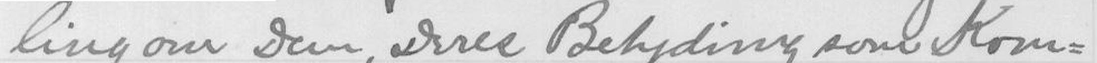ling om Dem, Deres Betydning som Kom-
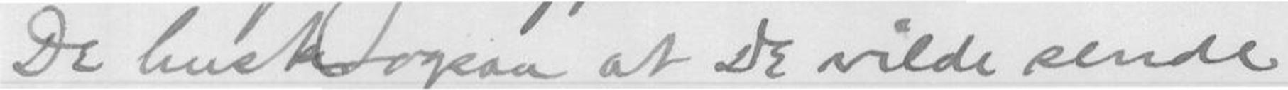De husker ogsaa at De vilde sende
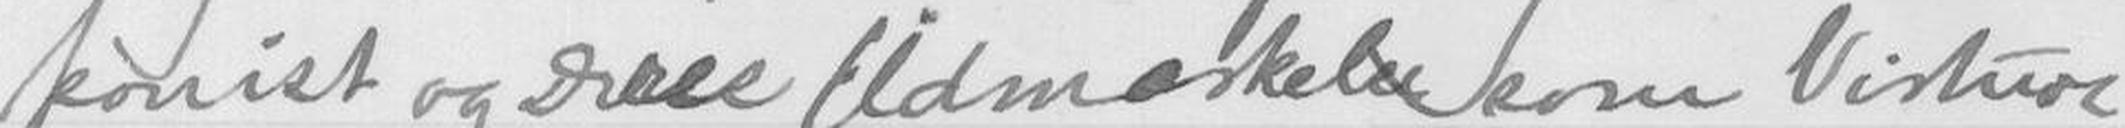ponist og Deres Udmærkelse som Vistnok
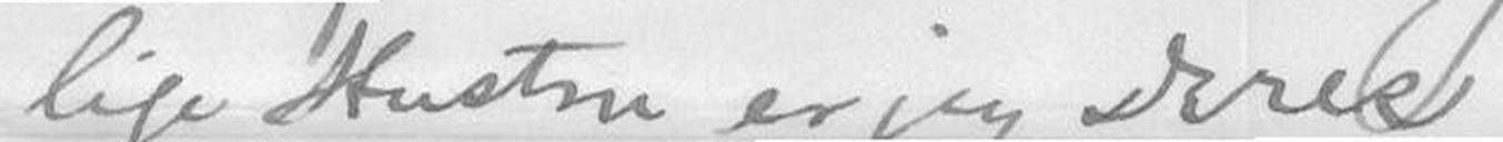lige Hustru er jeg Deres
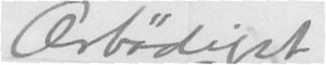Ærbødigst
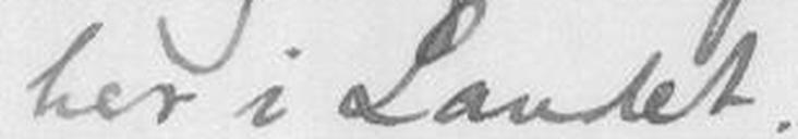her i Landet.
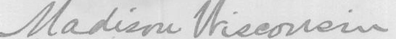Madison Wisconsin
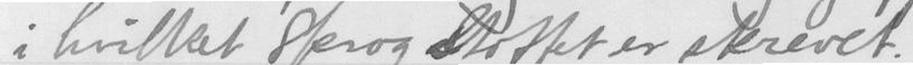i hvilket Sprog Stoffet er skrevet.
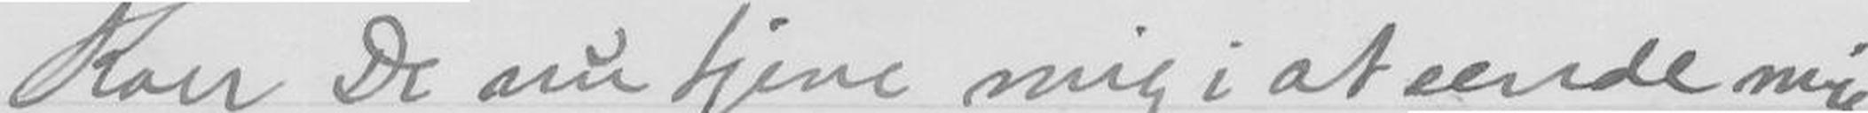Kan De nu tjene mig i at sende mig
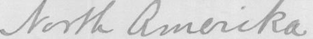North Amerika.
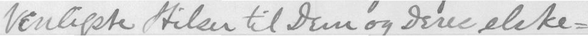Venligste Hilsen til Dem og Deres elske-
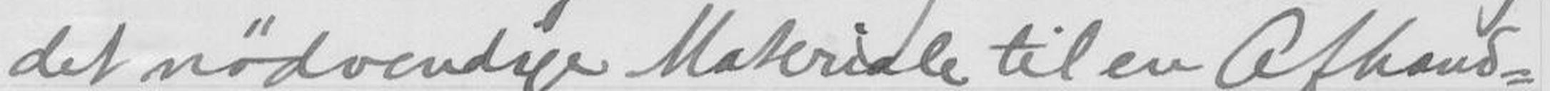det nødvendige Materiale til en Afhand-
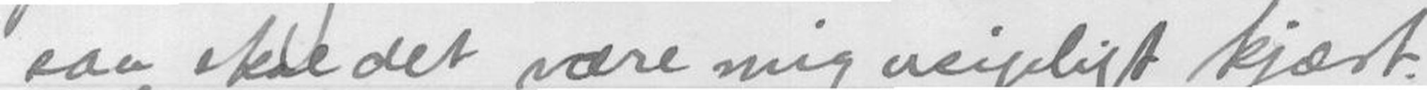saa skal det være mig kjært.
